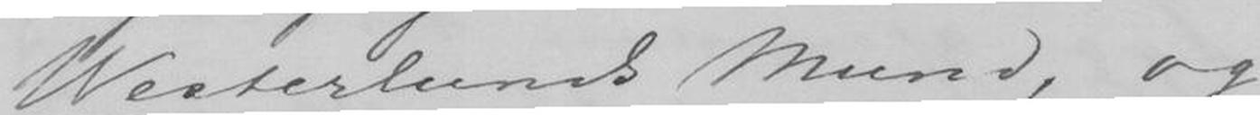Westerlunds Mund, og
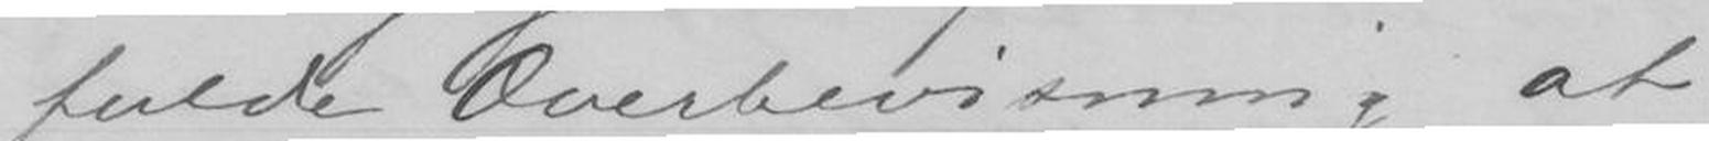fulde Overbevisning at
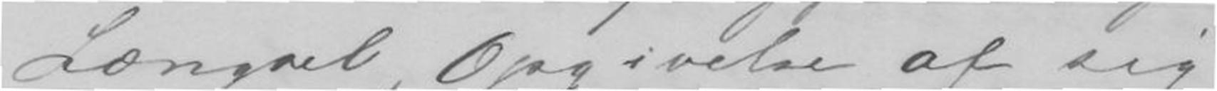Længsel, Opgivelse af sig
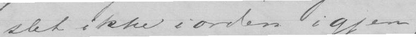slet ikke i orden igjen
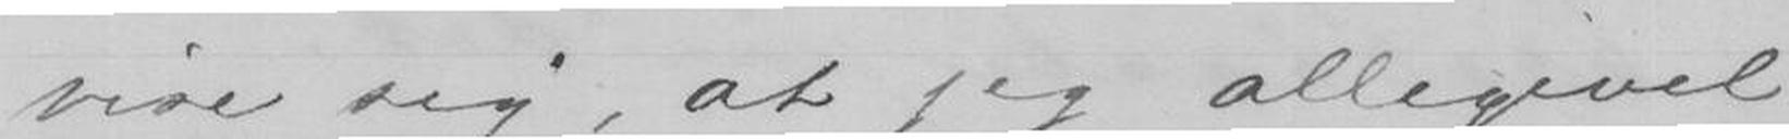vise sig, at jeg alligevel
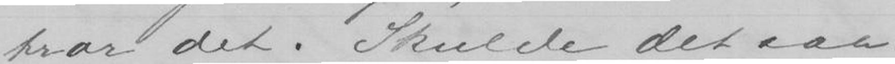tror det. Skulde det saa
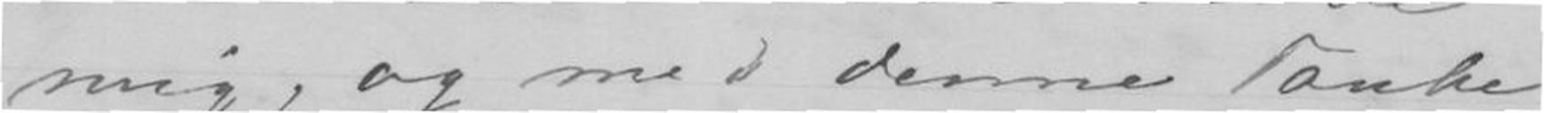mig, og med denne Tanke
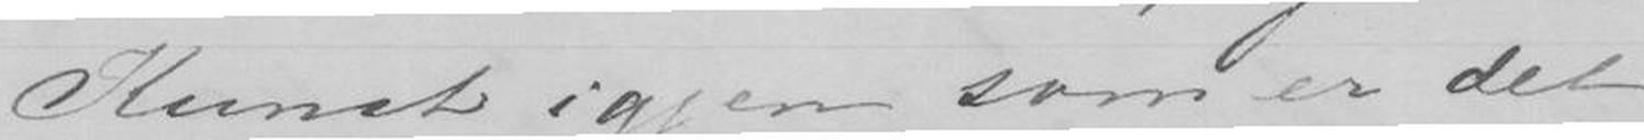Kunst igjen, som er det
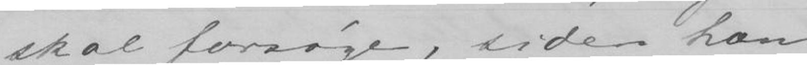skal forsøge, siden han
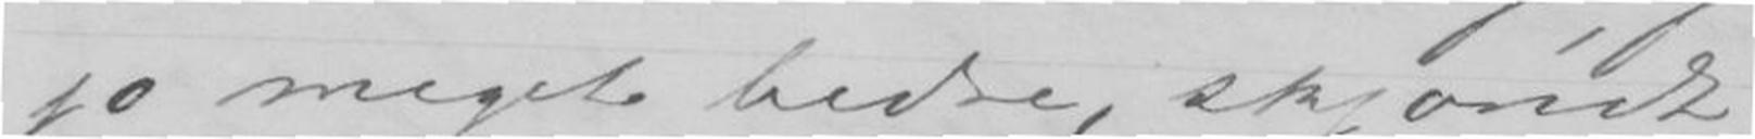jo meget bedre, skjøndt
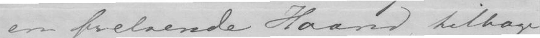en frelsende Haand, tilbake
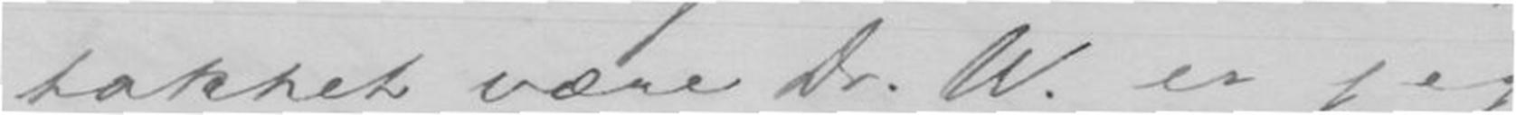takket være Dr. W. er jeg
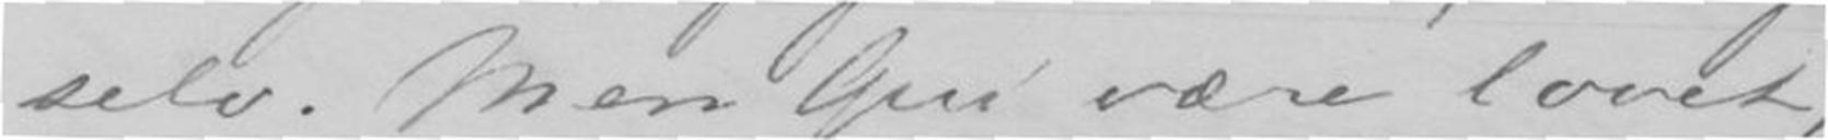selv. Men Gud være lovet,
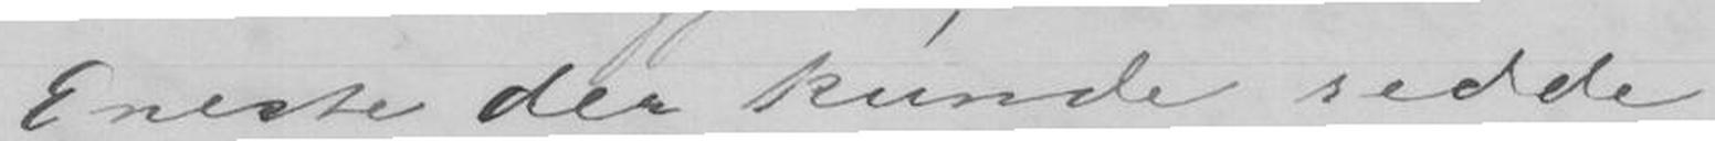Eneste der kunde redde
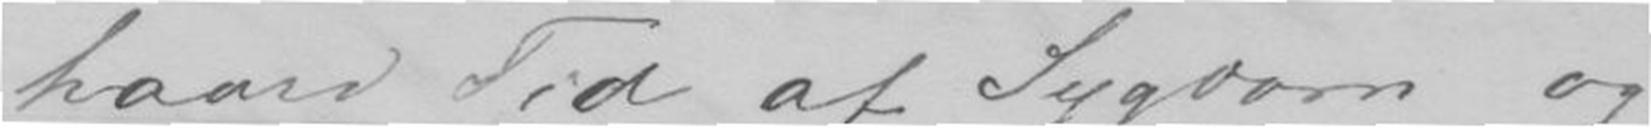haard Tid af Sygdom og
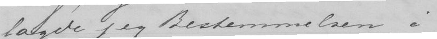lagde jeg Bestemmelsen i
